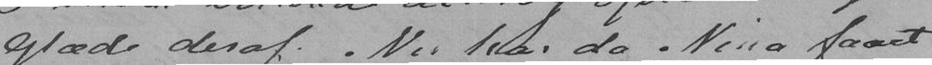glæde deraf. Nu har da Nina faaet
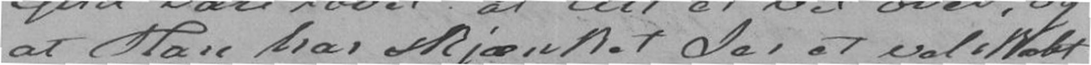at Han har skjænket Jer et velskabt
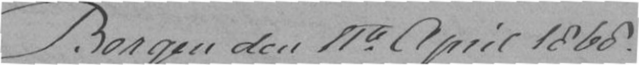Bergen den 11te April 1868.
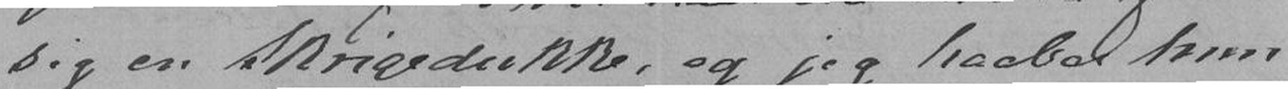sig en Skrigedukke, og jeg haaber hun
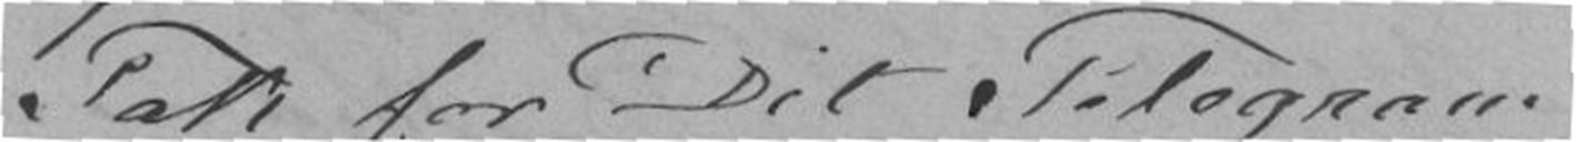Tak for Dit Telegram
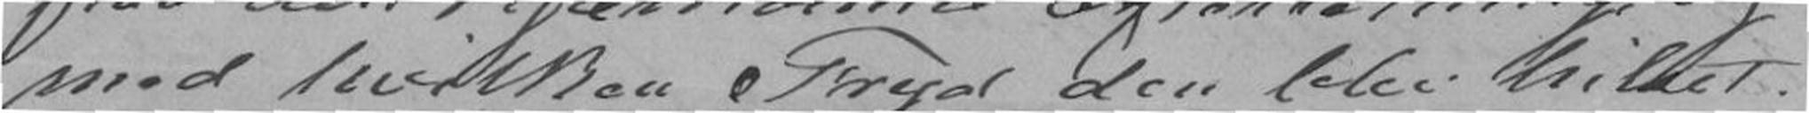med hvilken Fryd den blev hilset.
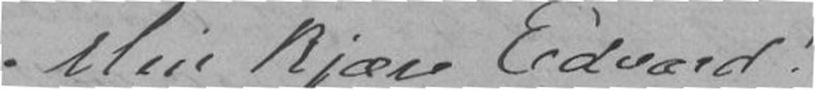Min kjære Edvard!
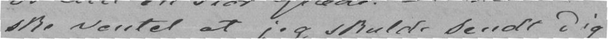ske ventet at jeg skulde sende Dig
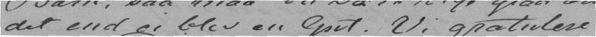det end ei blev en Gut. Vi gratulere
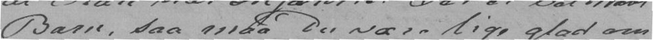Barn, saa maa Du være lige glad om
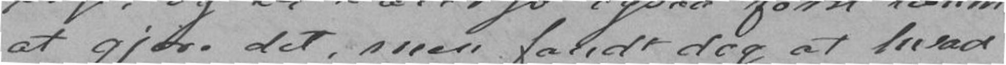at gjøre det, men fandt dog at hvad
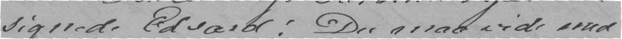signede Edvard! Du maa vide med
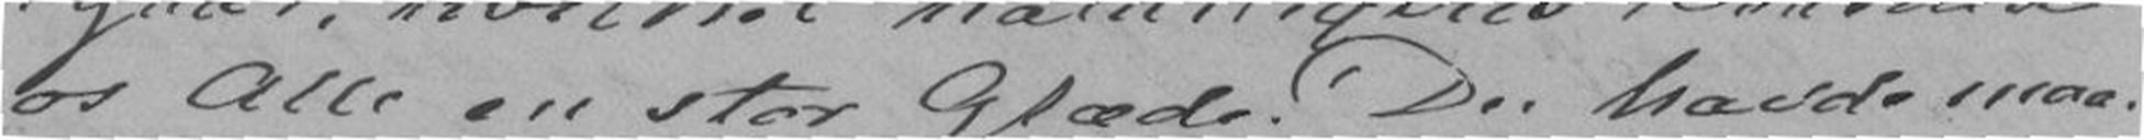os Alle en stor Glæde. Du havde maa-
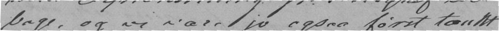bage, og vi være jo ogsaa først tænkt
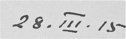28. III. 15.
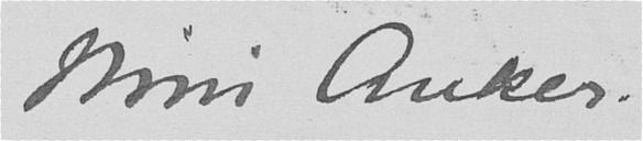Nini Anker
